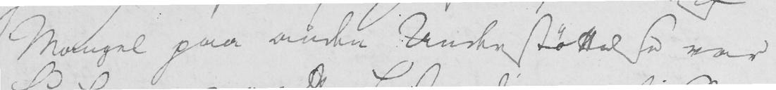Mangel paa anden Understøttelse var
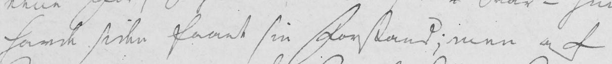havde siden faaet sin Forstand; men af
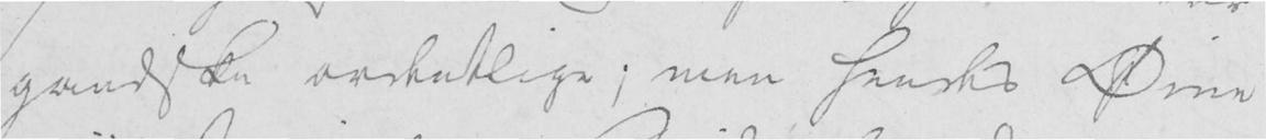gandske ordentlige; men hendes Øine
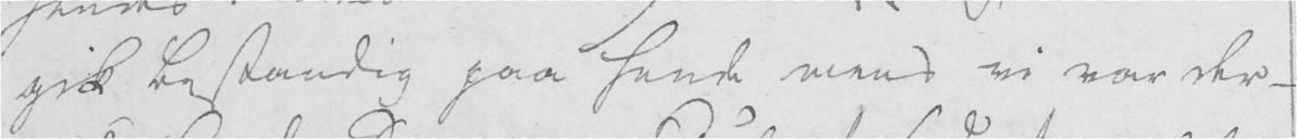gik bestandig paa hende mens vi var der -
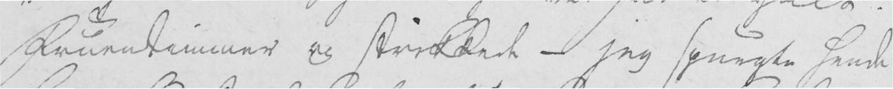Fruentimmer og strikkede - jeg spurgte hende
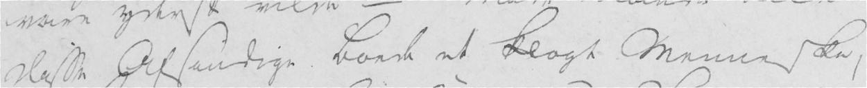disse Afsindige boede et blegt Menneske,
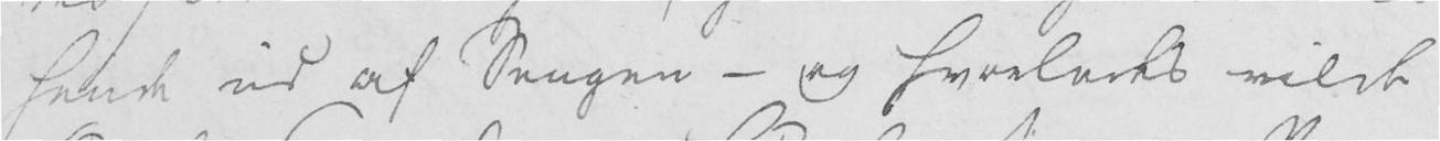hende ud af Sengen - og hvorledes vilde
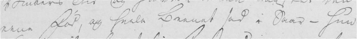eene Fod, og heele Beenet sad i Saar - hun
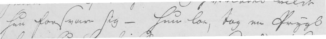hun forsvare sig - hun loe, tog en Prygl
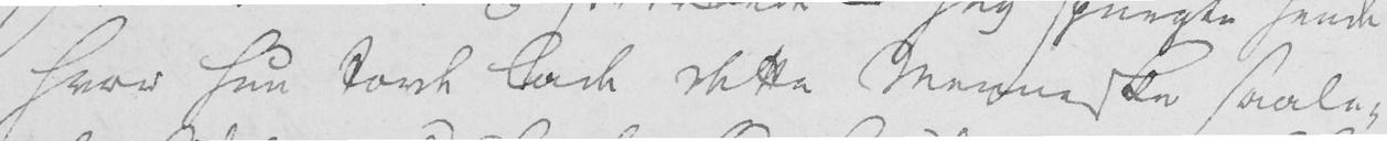hvor hun torde lade dette Menneske saale-
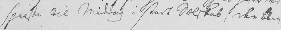spiiste til Middag i stort Sælskab, der blev
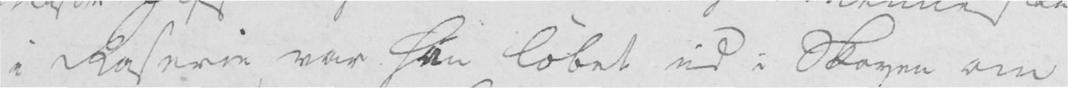i Raserie var hun løbet ud i Skoven om
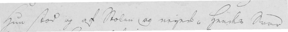hun stod op af Stolen og neyet; Hendes Svar
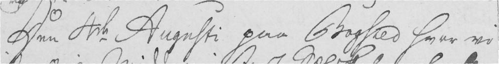Den 4de Augusti paa Bogsted hvor vi
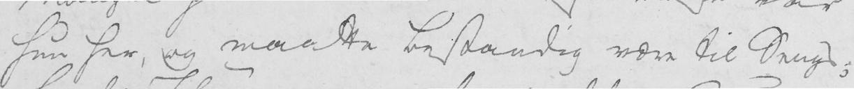hun her, og maatte bestandig være til Sengs;
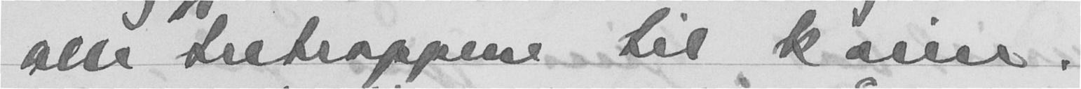alle tretrappene til kaien.
